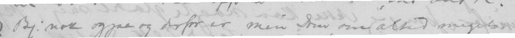Bj. nok ogsaa og derfor er min Dom om altid meget
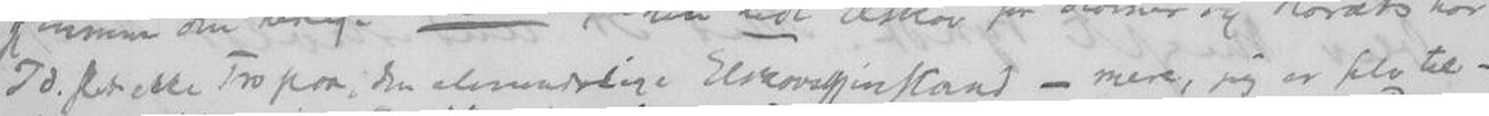Id. slet ikke Tro paa den almindelige Elskovsgjenstand - mere, jeg er selv til-
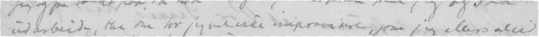udarbeide, thi den har jeg vel ikke imporvisert, som jeg ellers altid
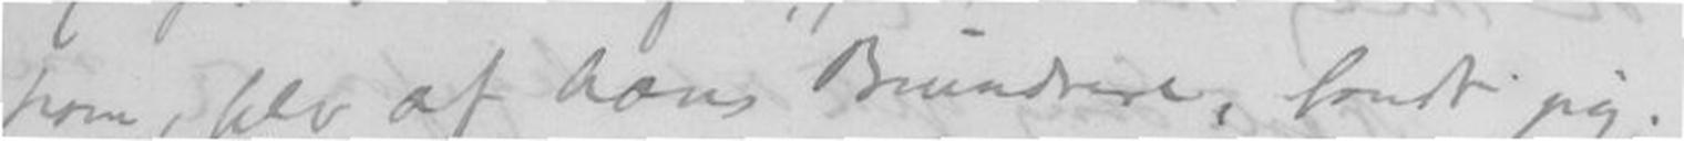ham, selv af hans Beundere, fandt jeg.
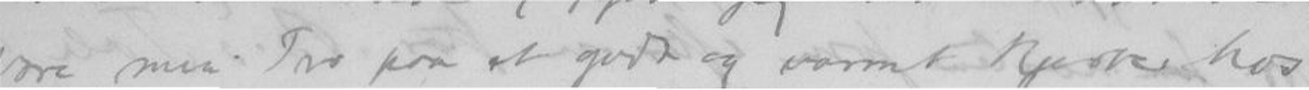være maa. Tro paa at godt og varmt Hjerte hos
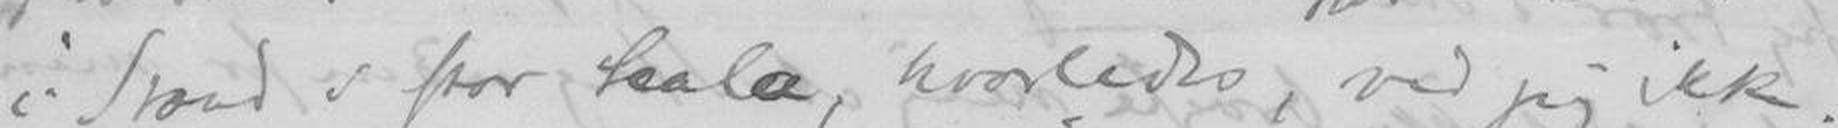i Stand i stor Scala, hvorledes, ved jeg ikke.
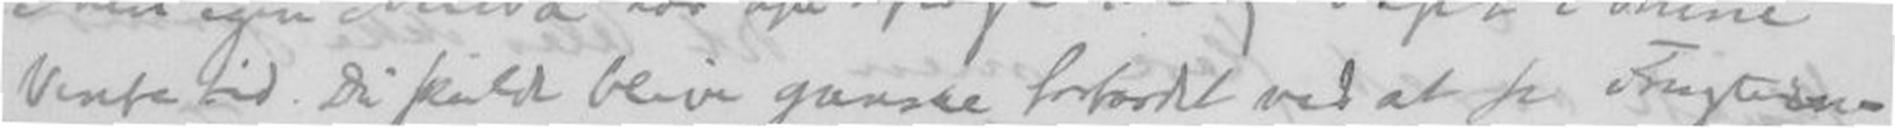Ventetid Du skulde blive ganske forfærdet ved at se Frugten
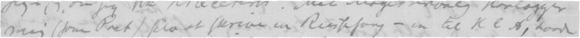mig (som Poet) selv at skrive en Russesang - en til H C A, havde
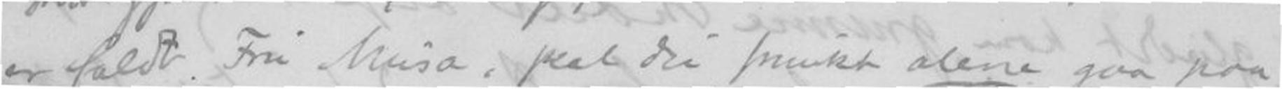er faldt. Fru Musa, skal du smukt alene gaa paa
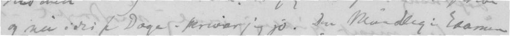og nu i disse Dage - skriver jeg jo. Den Mundleg i Examen
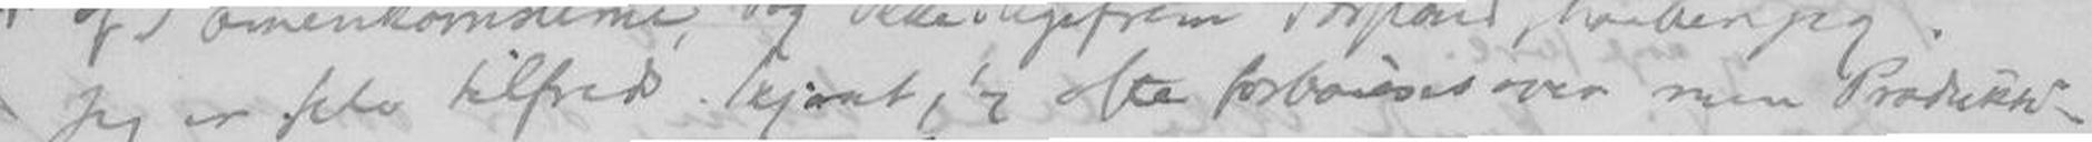Jeg er selv tilfreds. Skjønt jeg ofte forbauses over min Produkti-
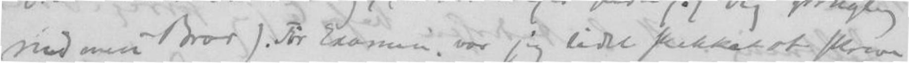med min Bror). For Examen var jeg lidet skikket at skrive
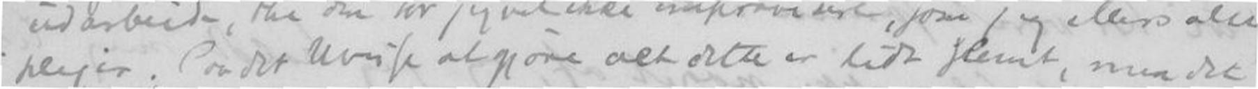pleier. Paa det Uvisse at gjøre alt dette er lidt slemt, men det
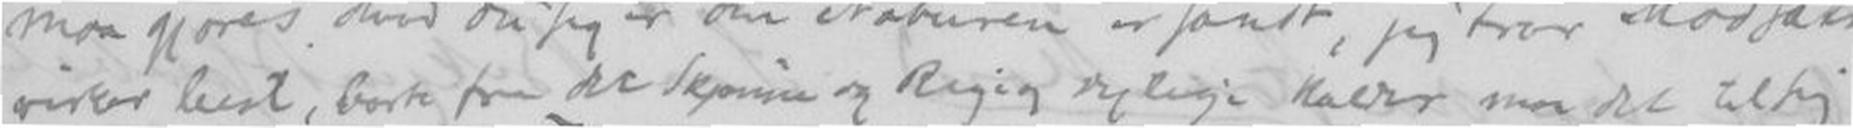virker best, borte fra det Skjønne og Rige og dejlige holder man det tilsig
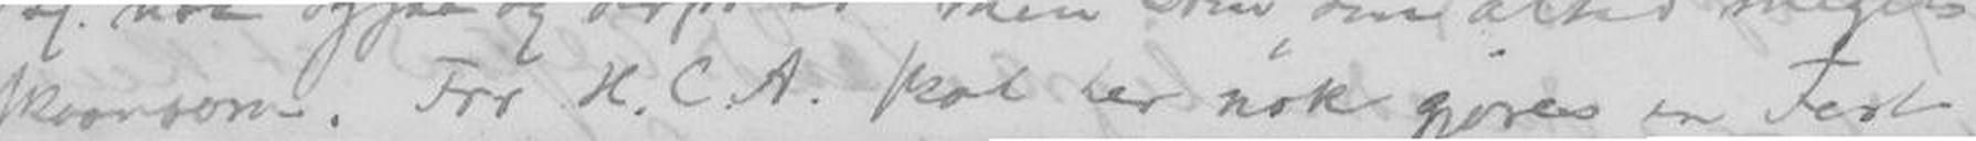skonsom. For H. C. A. skal her nok gjøre en Fest
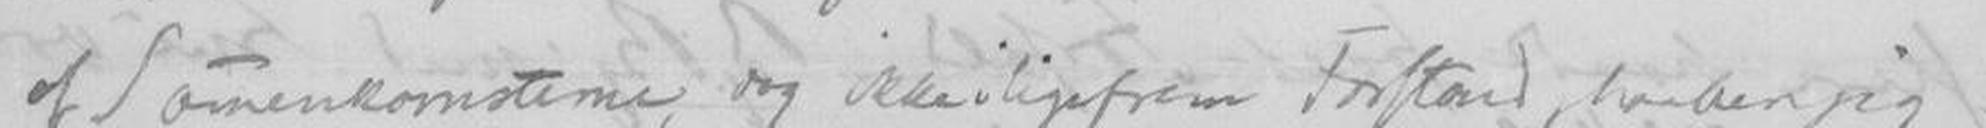af Samenkomsterne, dog ikke ligefrem Forstand, tænker jeg.
